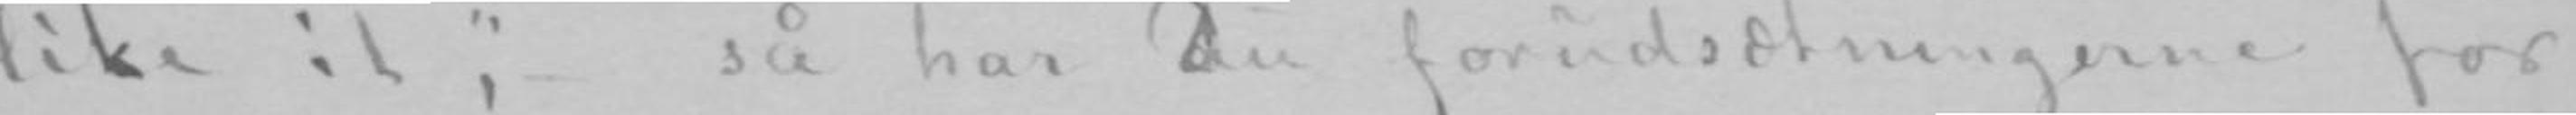like it»,- så har Dudu forudsætningerne for
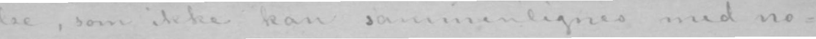værelse, som ikke kan sammenlignes med no-
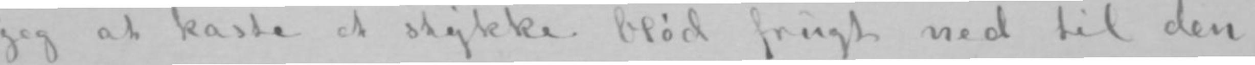jeg at kaste et stykke blød frugt ned til den,
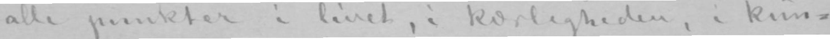alle punkter i livet, i kærligheden, i kun-
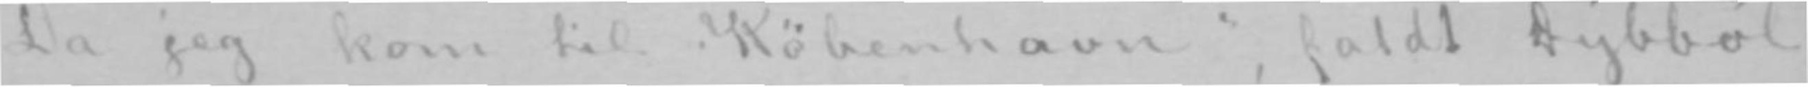Da jeg kom til København, faldt Dybbøl.
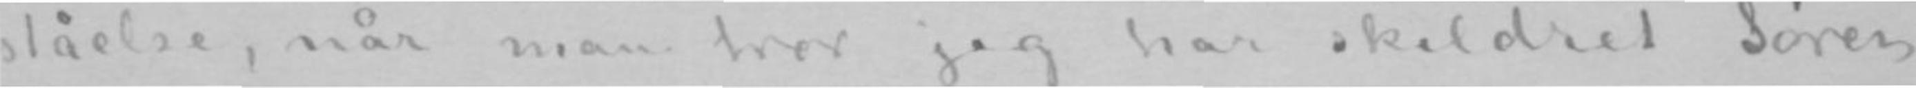ståelse, når man tror jeg har skildret Søren
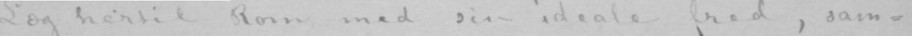Læg hertil Rom med sin ideale fred, sam-
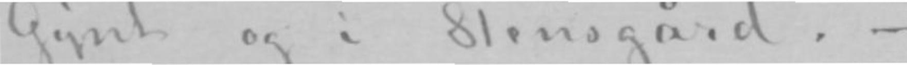Gynt og i Stensgård.-
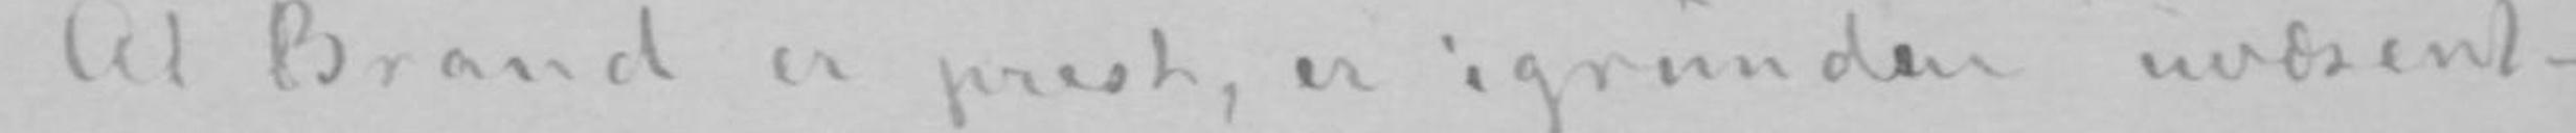dre.) At Brand er prest, er igrunden uvæsent-
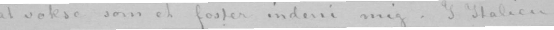at vokse som et foster indeni mig. I Italien
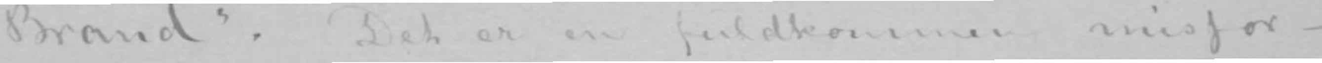«Brand». Det er en fuldkommen misfor-
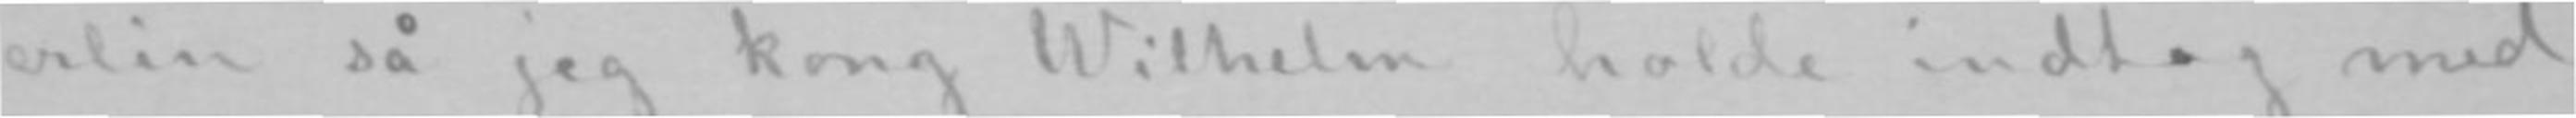I Berlin så jeg kong Wilhelm holde indtog med
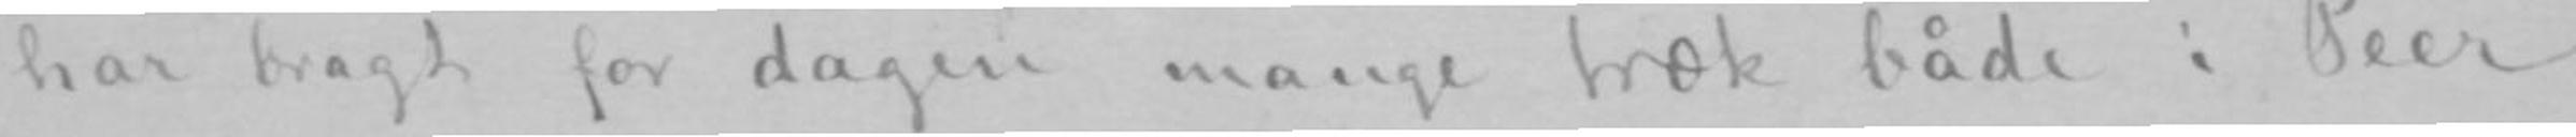har bragt for dagen mange træk både i Peer
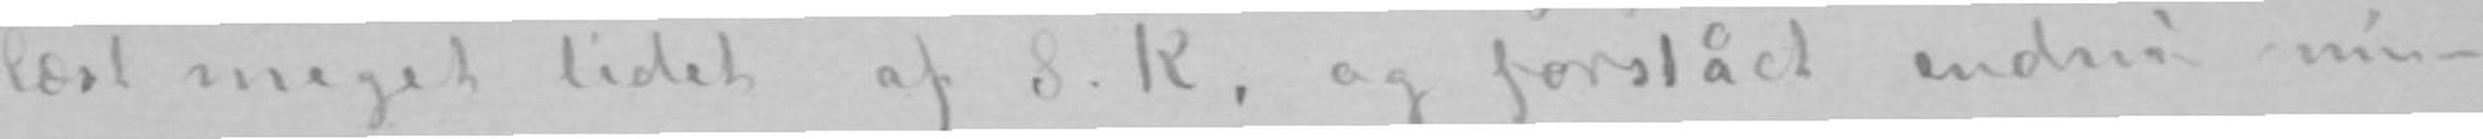læst meget lidet af S. K. og forstået endnu min-
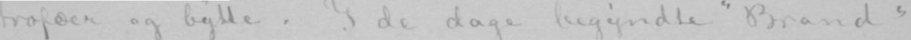trofæer og bytte. I de dage begyndte «Brand»
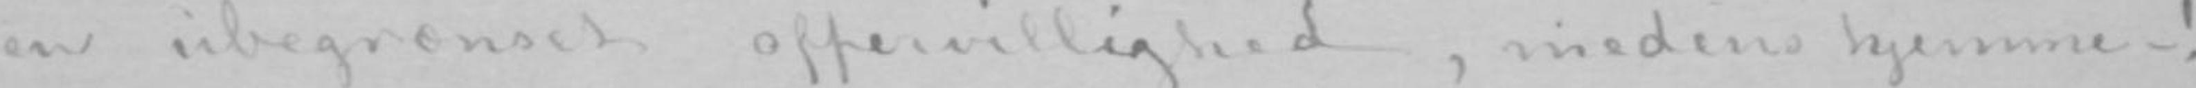en ubegrænset offervillighed, medens hjemme-!
